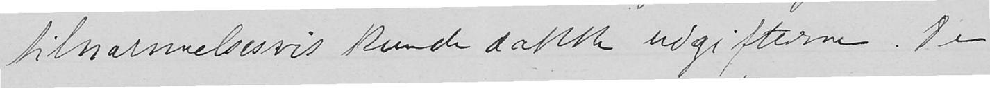tilnærmelsesvis kunde dække udgifterne. De
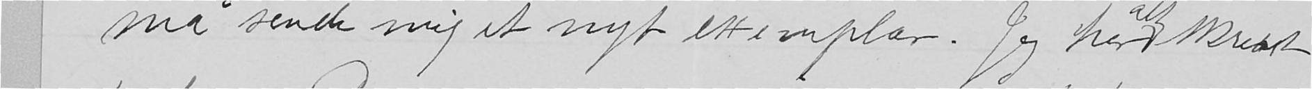må sende mig et nyt exemplar. Jeg har all skrevet
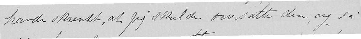havde skrevet, at jeg skulde oversætte den, og så
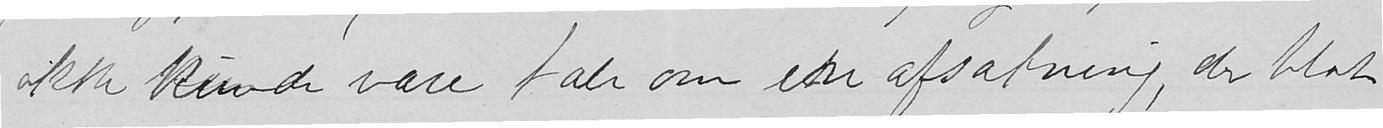ikke kunde være tale om en afsætning, der blot
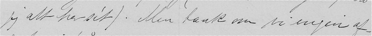jeg alt har sét). Men tænk om vi ingen af-
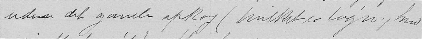uden det gamle opkog (hvilket er løgn, hvad
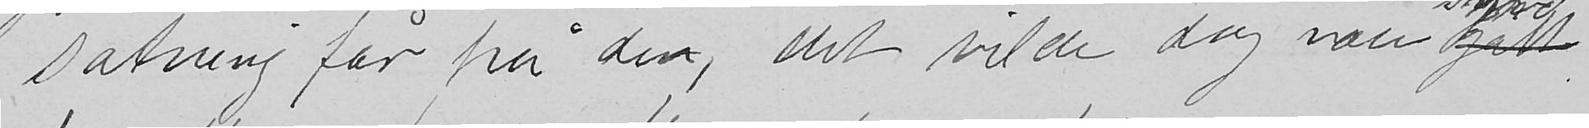sætning får på den, det vilde dog være godt
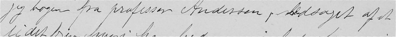jeg bogen fra professer Andersen, ledsaget af et
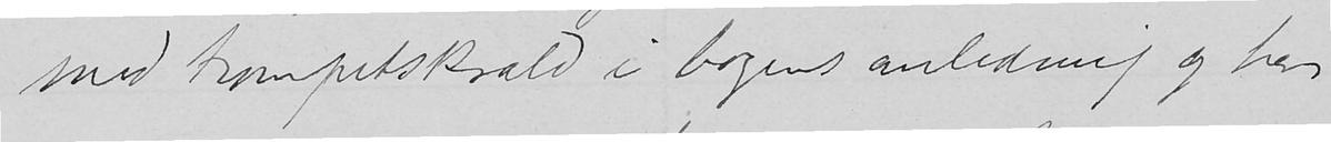med trompetskrald i bogens anledning og har
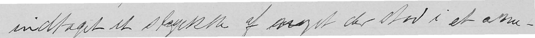indtaget et stykke af noget der stod i et ame-
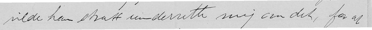vilde han strax underrette mig om det, for at
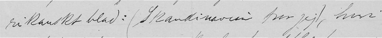rikanskt blad: (Skandinavien tror jeg), hvori
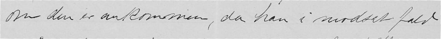om den er ankommen, da han i modsat fald
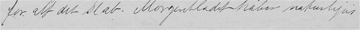for at det slæb. Morgenbladet håber naturligvis
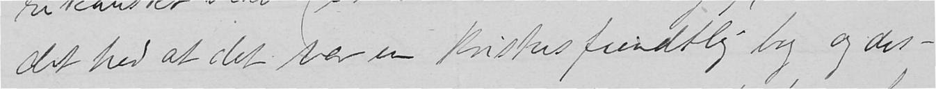det hed at det var en Kristusfiendtlig bog og des-
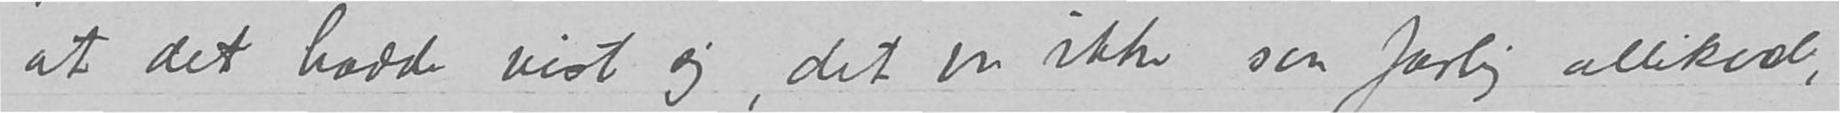at det hadde vist sig, det vaer ikke saa farlig allikevel,
